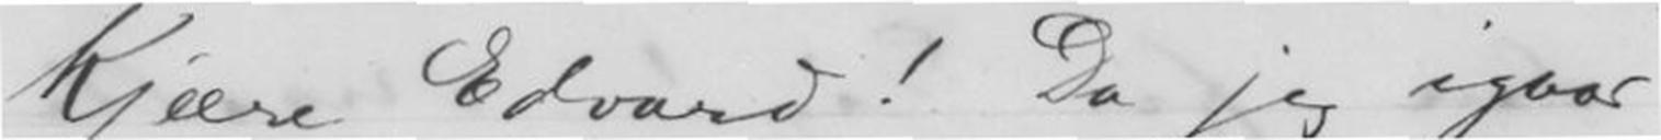Kjære Edvard! Da jeg igaar
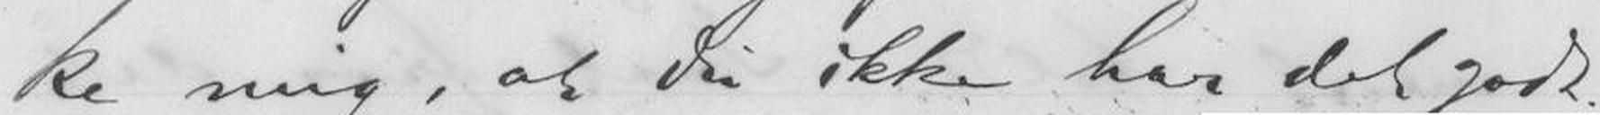ke mig, at Du ikke har det godt.
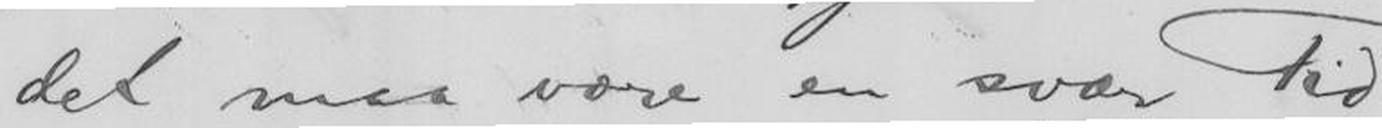det maa være en svær Tid
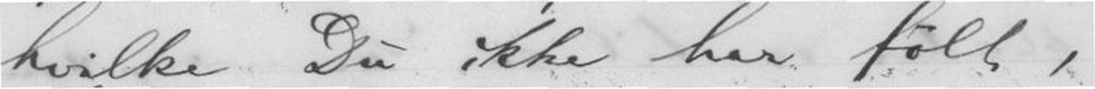hvilke Du ikke har følt,
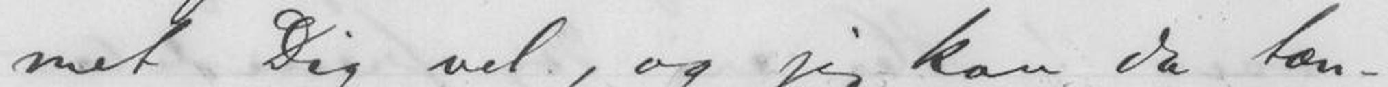met Dig vel, og jeg kan da tæn-
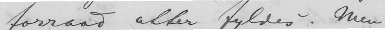forraad atter fyldes. Men
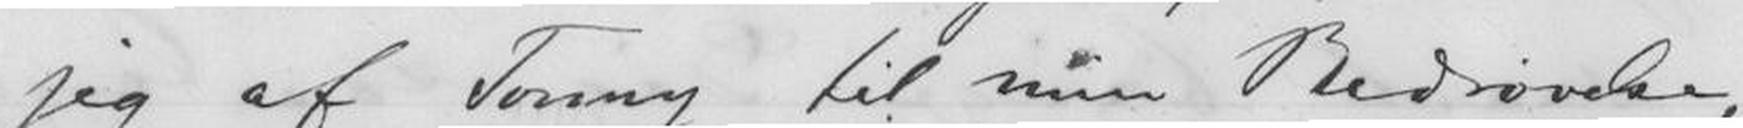jeg af Tonny til min Bedrøvelse,
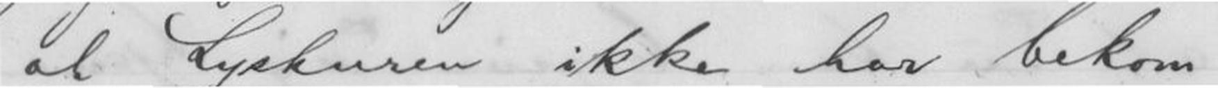at Lyskuren ikke har bekom-
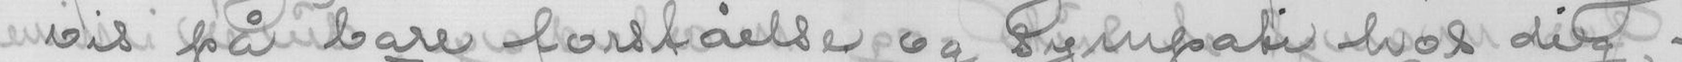vis på bare forståelse og sympati hos dig, -
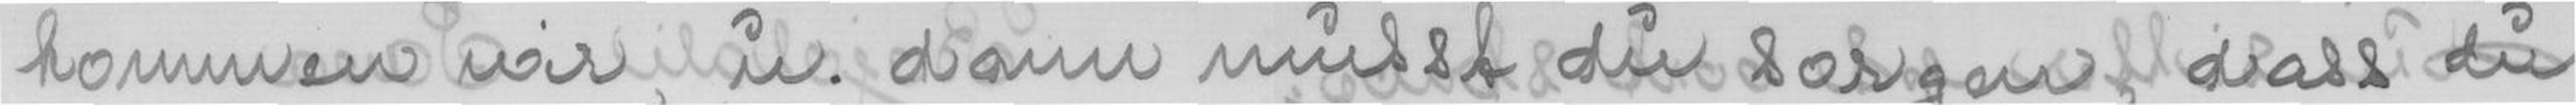kommen wir, u. dann musst du sorgen, dass du
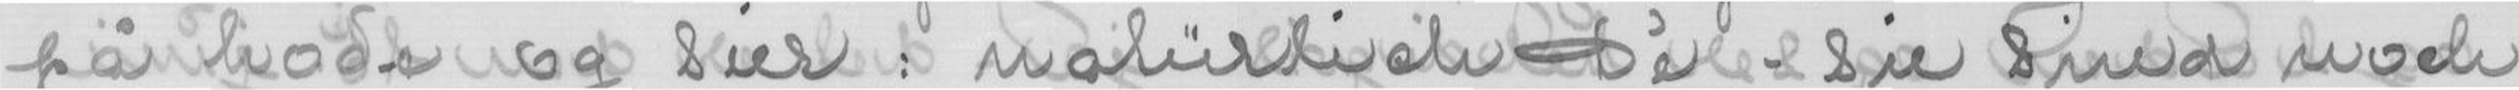på hode og sier: natürlich Dé - sie sind woch
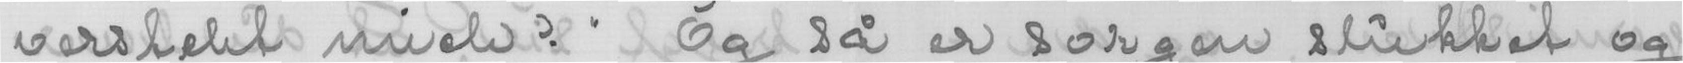versteht mich!" Og så er sorgen slukket og
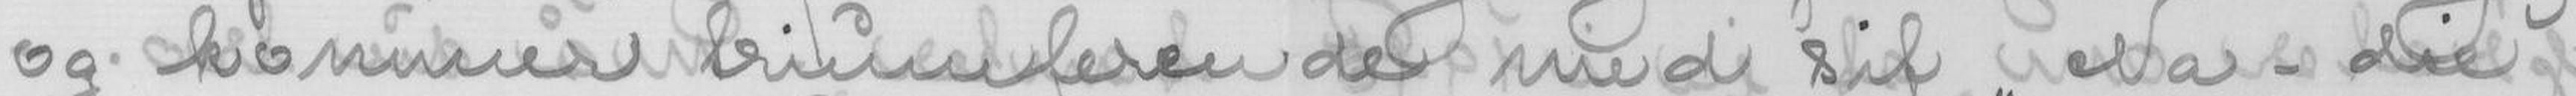og kommer triumferende med sit "Na - die
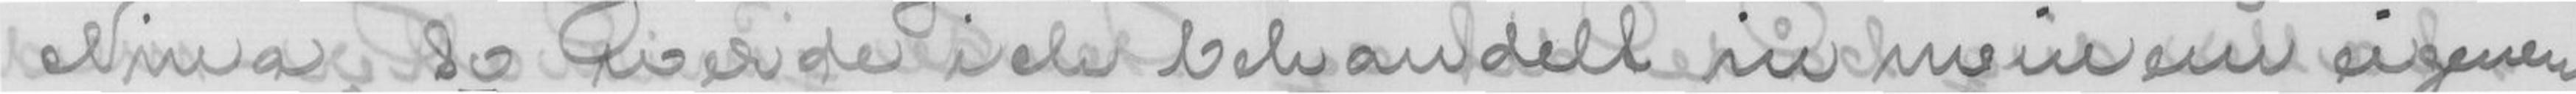Nina, so werde ich behandelt in meinem eigenen
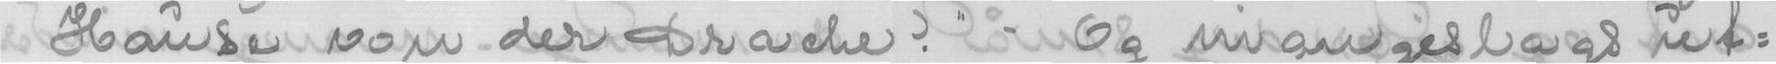Hause von der Drache!" - Og mangeslags ut-
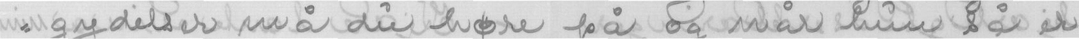gydelser må du høre på, og når hun så er
